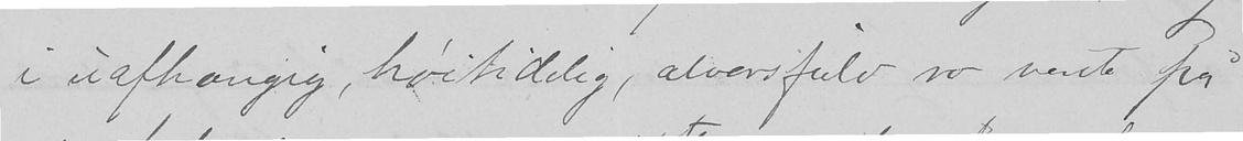i uafhængig, høitidelig, alvorsfuld ro vente på
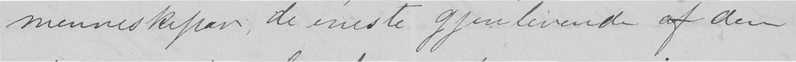menneskepar, de eneste gjenlevende af den
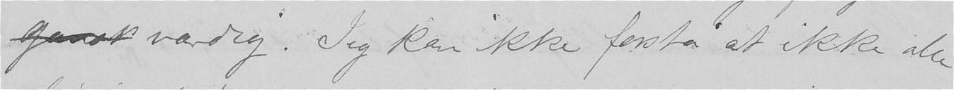gansk værdig. Jeg kan ikke forstå at ikke alle
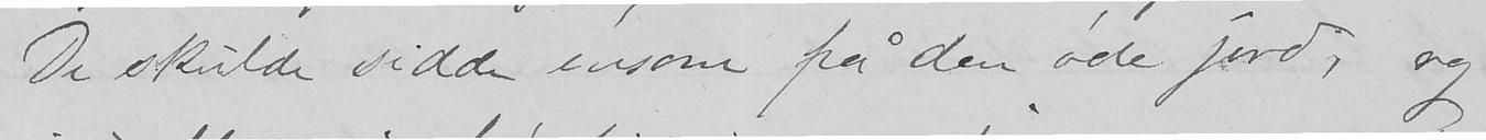De skulde sidde ensom på den øde jord, og
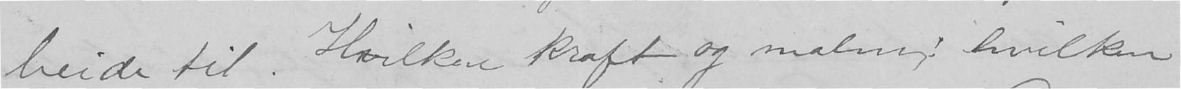beide til. Hvilken kraft og malm! hvilken
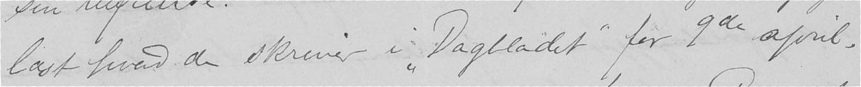læst hvad de skriver i "Dagbladet" for 9de april.
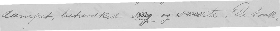dæmpet, behersket sorg og smerte. De træk-
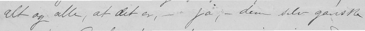alt og alle, at det er, - ja; - dem selv ganske
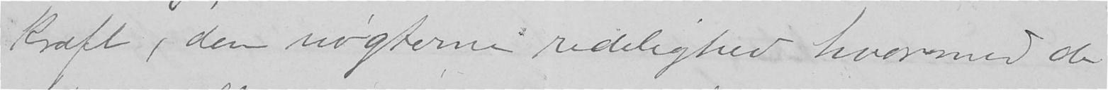kraft, den nøgterne redelighed, hvormed de
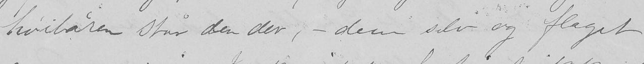høibåren står den der, - dem selv og flaget
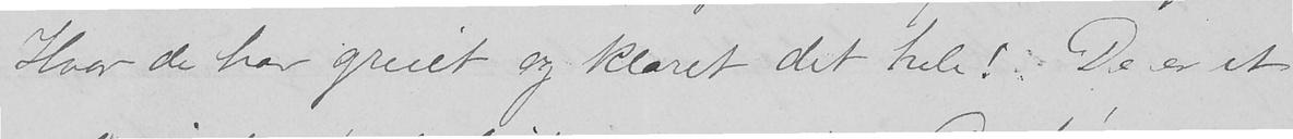Hvor de har greiet og klaret det hele! De er et
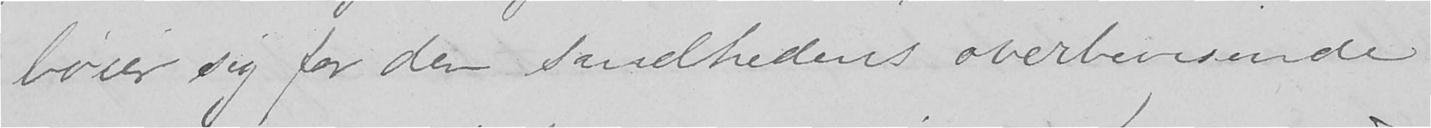bøier sig for den sandhedens overbevisende
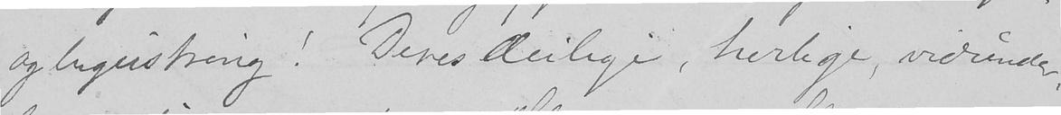og begeistring! Deres deilige, herlige, vidunder-
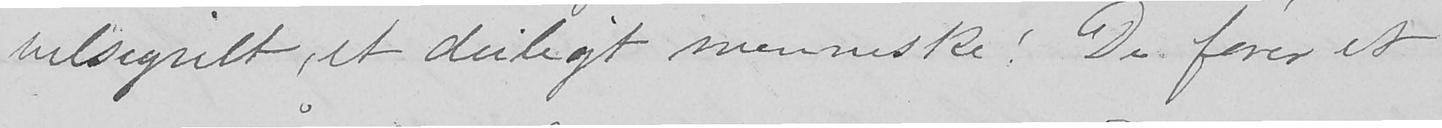velsignet, et deiligt menneske! De fører et
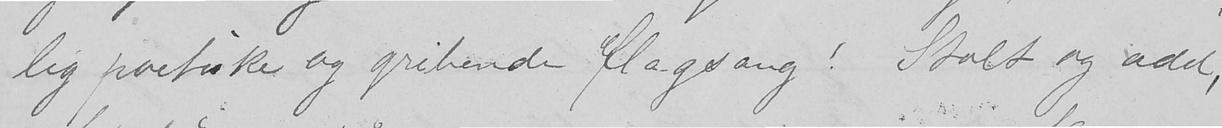lig poetiske og gribende flagsang! Stolt og ædel,
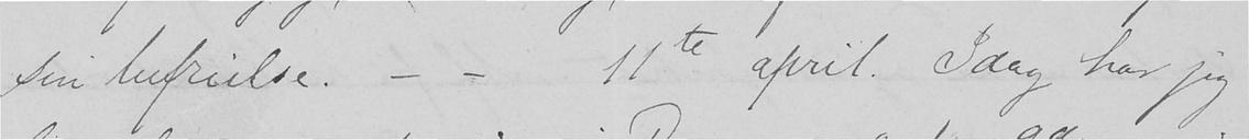sin befrielse. - -  11te april. Idag har jeg
